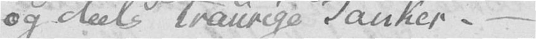og deels traurige Tanker. –
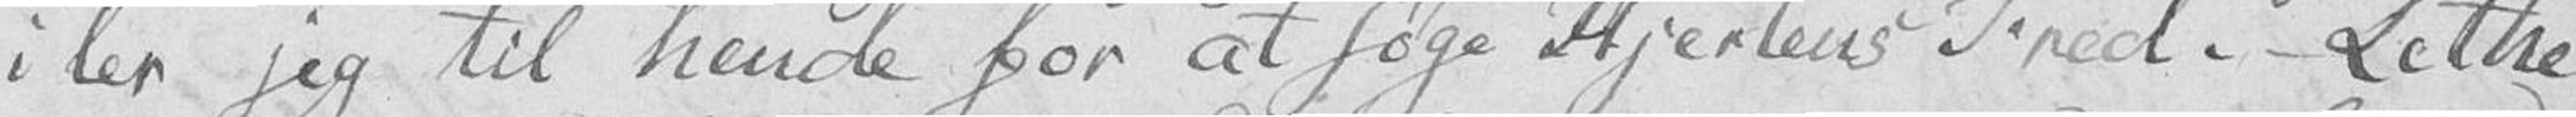iler jeg til hende for at søge Hjertens Fred. – Lethe
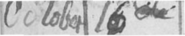October 16de
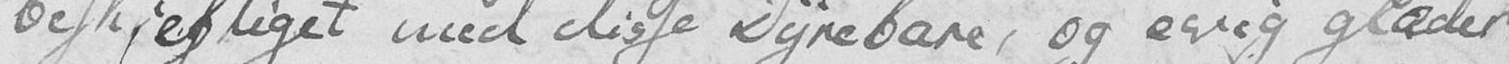beskjeftiget med disse Dyrebare, og evig glæder
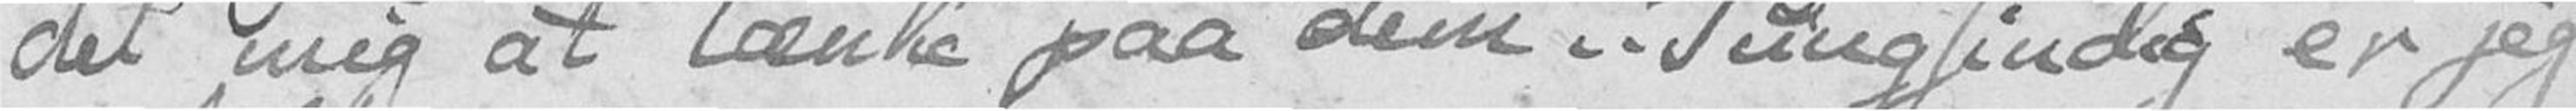det mig at tænke paa dem. . Tungsindig er jeg
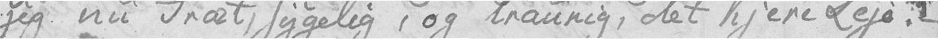jeg nu Træt, sygelig, og traurig, det kjere Leje.
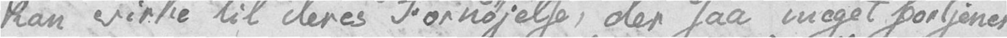kan virke til deres Fornøjelse, der saa meget fortjener
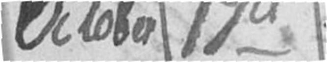October 17d
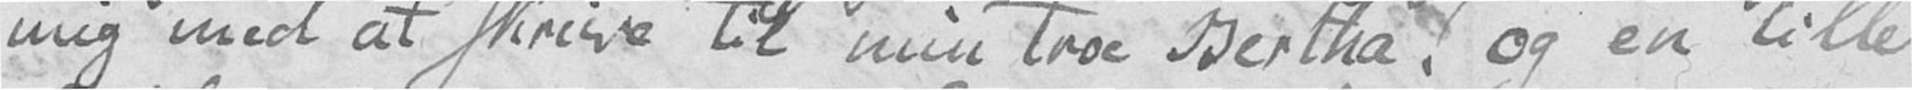mig med at skrive til min troe Bertha! og en lille
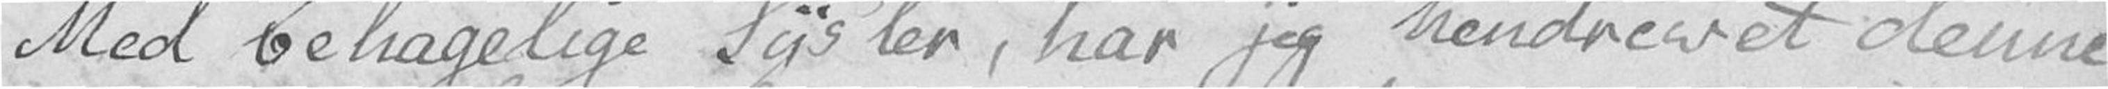Med behagelige Sysler, har jeg hendrevet denne
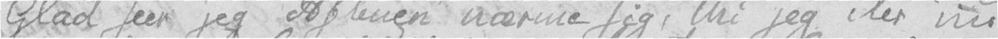Glad seer jeg Aftenen nærme sig, thi jeg iler nu
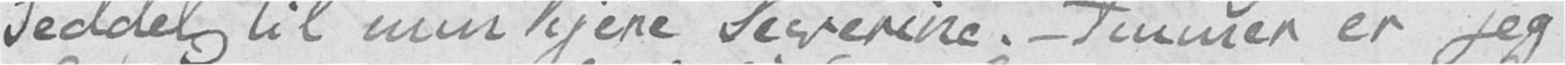Seddel til min kjere Severine. – Immer er jeg
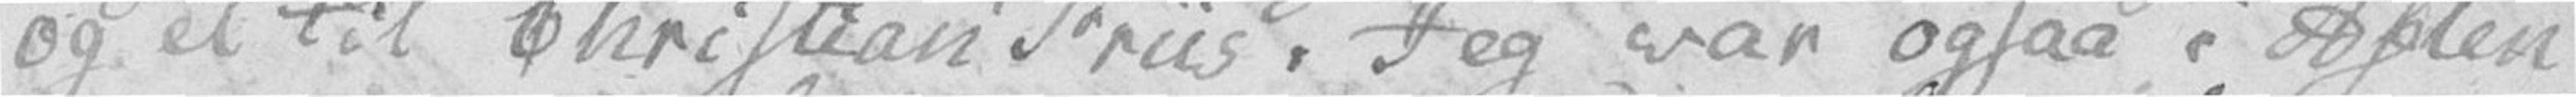og et til Christian Friis. Jeg var ogsaa i Aften
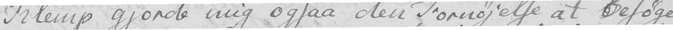Klemp gjorde mig ogsaa den Fornøjelse at besøge
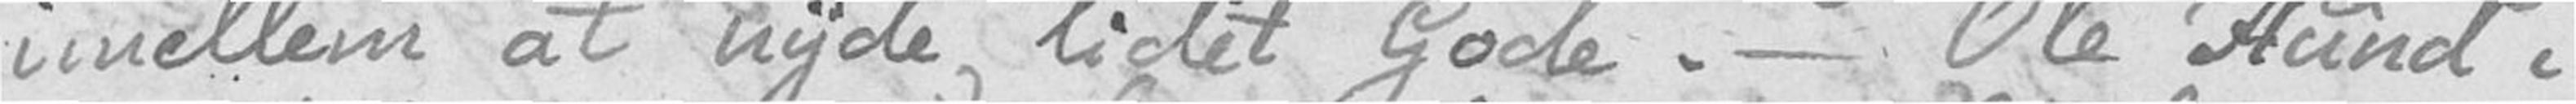imellem at nyde lidet Gode. – Ole Hund i
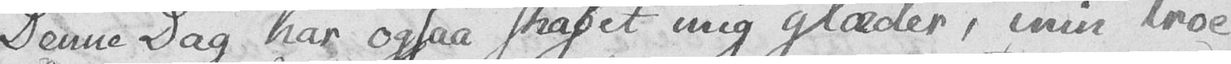Denne Dag har ogsaa skafet mig glæder, min troe
